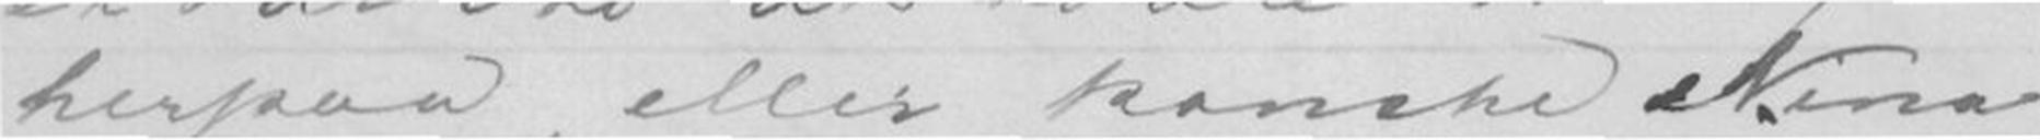herpaa, eller kanske Nina
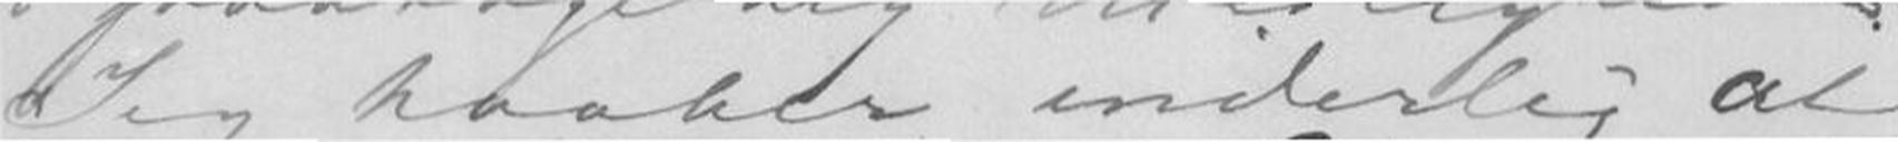Jeg haaber inderlig at
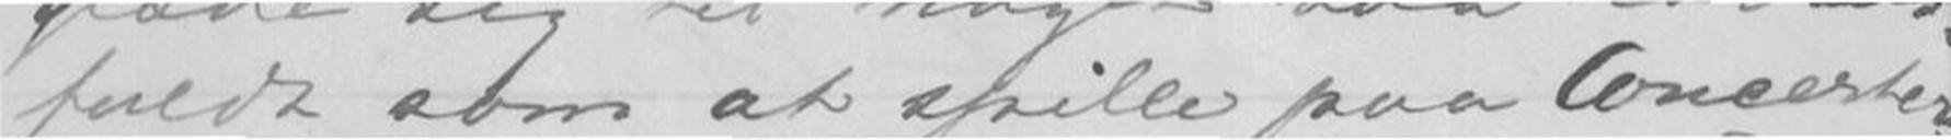fuldt som at spille paa Concerter,
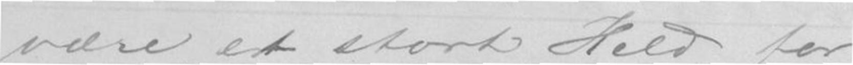være et stort Held for
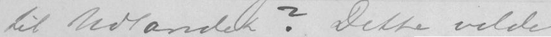til Udlandet? Dette vilde
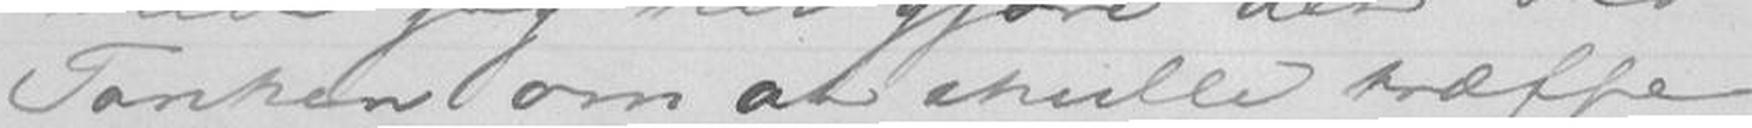Tanken om at skulle træffe
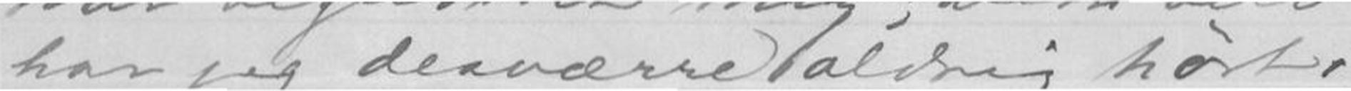har jeg desværre aldrig hørt i
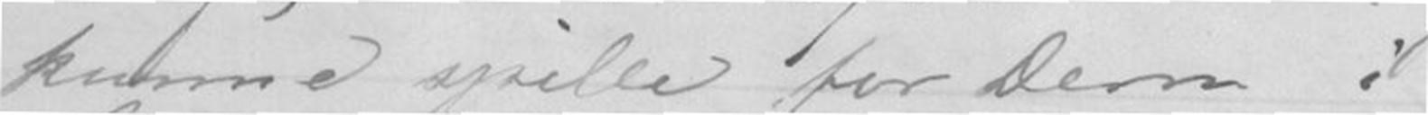kunne spille for Dem i
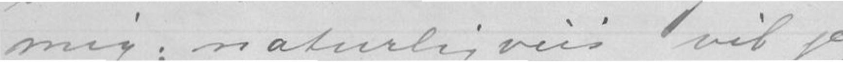mig; naturligvis vil jeg
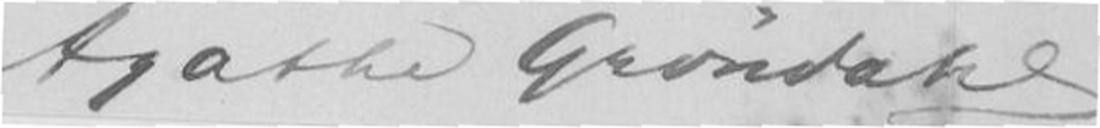Agathe Grøndahl
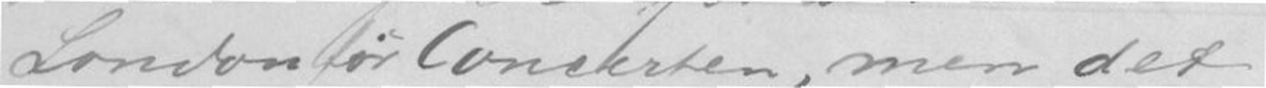London før Concerten, men det
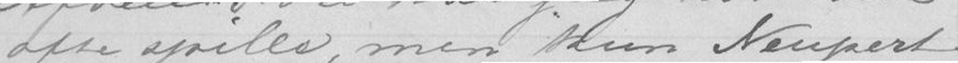ofte spilles, men kun Neupert
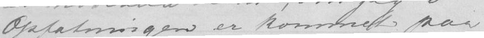Opfatningen er kommet paa
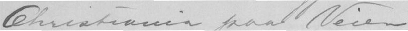Christiania paa Veien
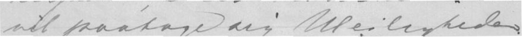vil paatage sig Uleiligheden.
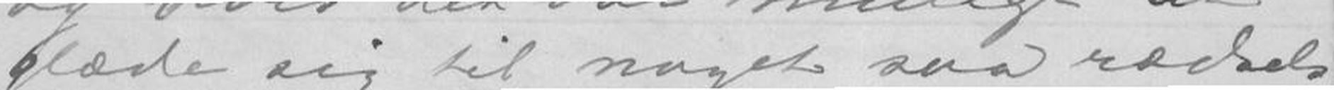glæde sig til noget saa rædsels-
